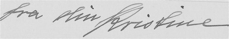fra din Kristine
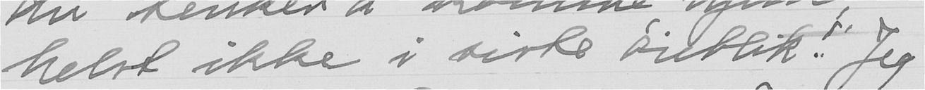helst ikke i siste øieblik! Jeg
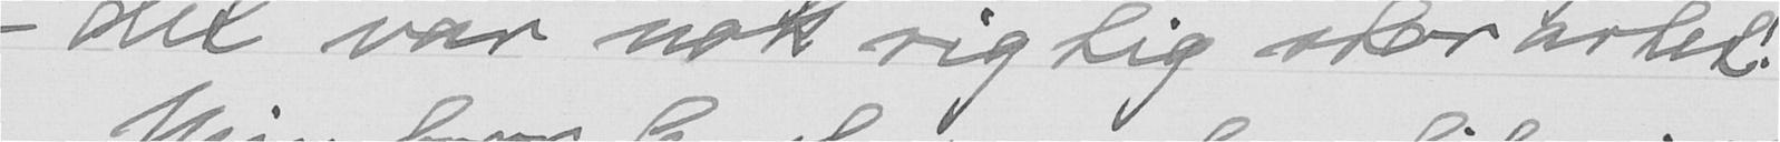- det var nok rigtig storartet!
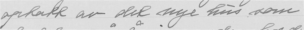optatt av det nye hus som
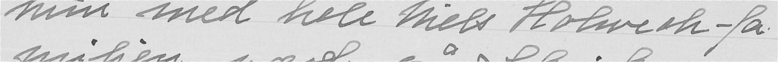hun med hele Niels Holsverk-fa
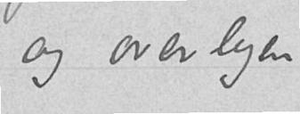og overlegen.
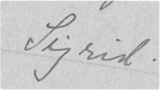Sigrid.
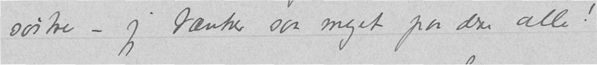søstre – jeg tænker saa meget paa dere alle!
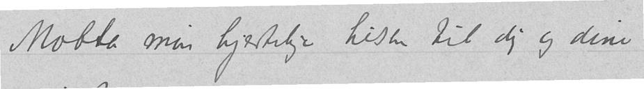Motta min hjertelige hilsen til dig og dine
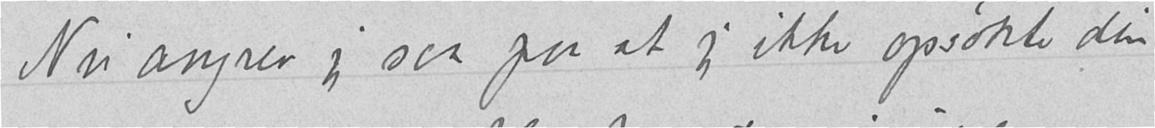Nu angrer jeg saa paa at jeg ikke opsøkte din
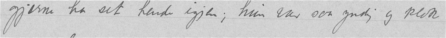gjerne ha set hende igjen; hun var saa yndig og klok
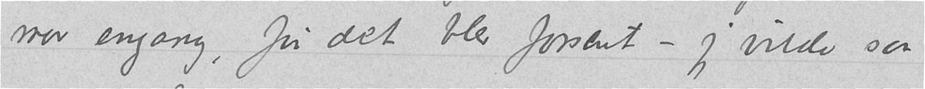mor engang, før det blev forsent – jeg vilde saa
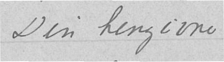Din hengivne
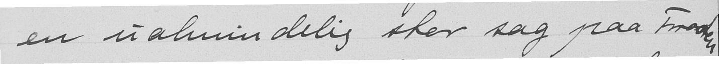en ualmindelig stor sag paa Frosten,
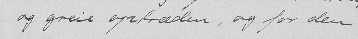og greie optræden, og for den
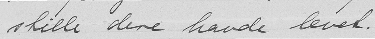stille dere havde levet.
